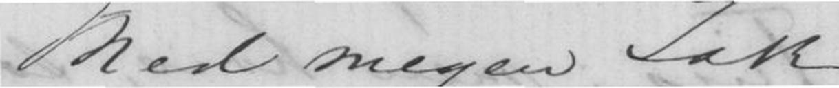Med megen Tak
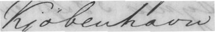Kjøbenhavn
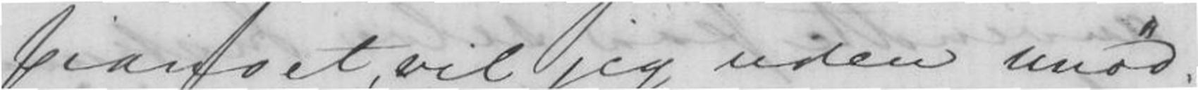pianoet, vil jeg uden unød
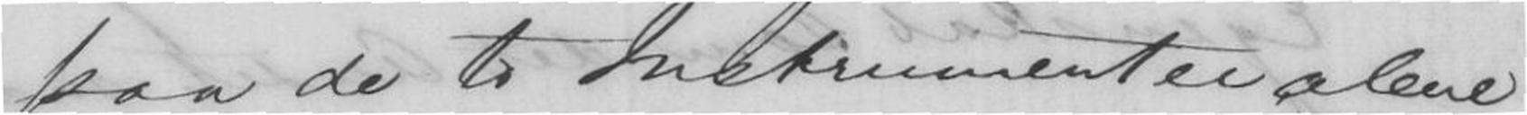paa de to Instrumenter alene
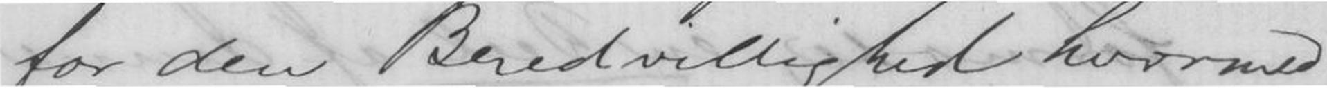for den Beredvillighed hvormed
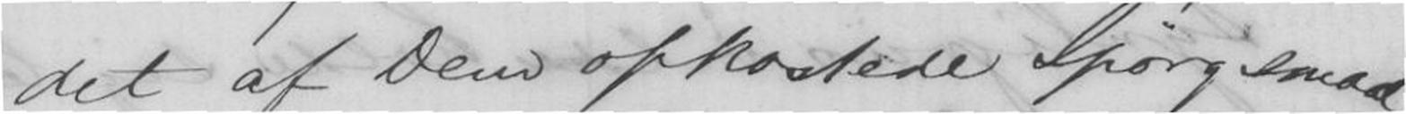det af Dem opkostede Spørgsmaal.
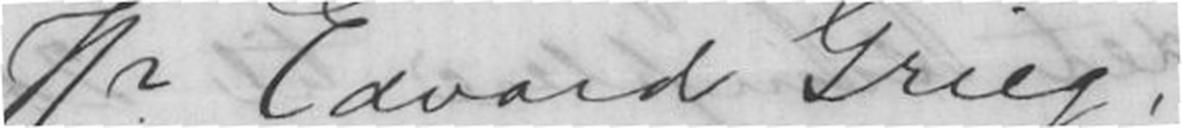Hr Edvard Grieg,
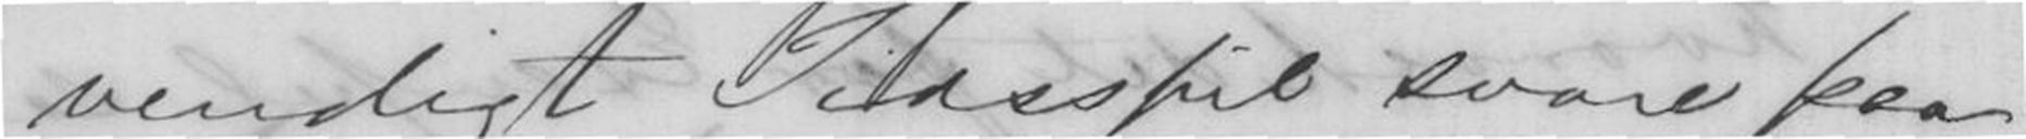venligt Tidsspil svare paa
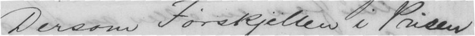Dersom Forskjellen i Prisen
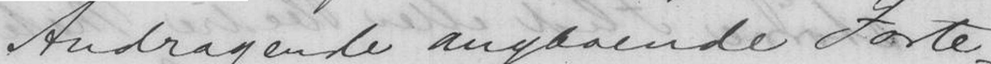Andragende angaaende Forte-
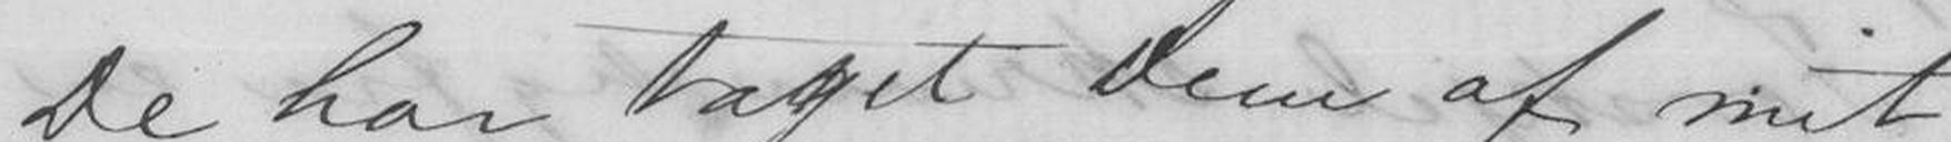De har taget Dem af mit
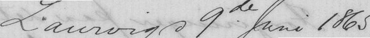Laurvigs 9de Juni 1865.
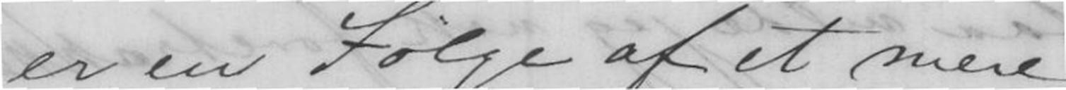er en Følge af et mere
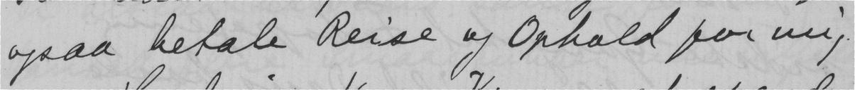ogsaa betale Reise og Ophold for mig.
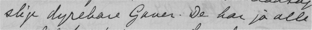slige dyrebare Gaver. De har jo alle
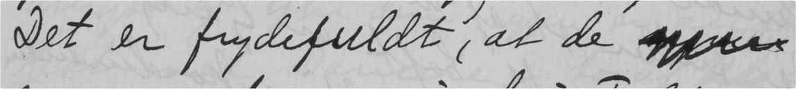Det er frydefuldt, at de
